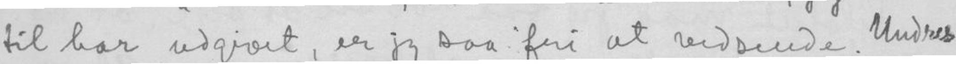til har udgivet, er jeg saa fri at vedsende. Undres
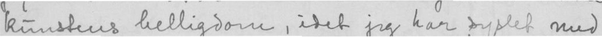kunstens helligdom, idet jeg har syslet med
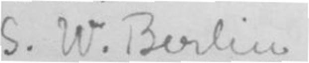S. W. Berlin
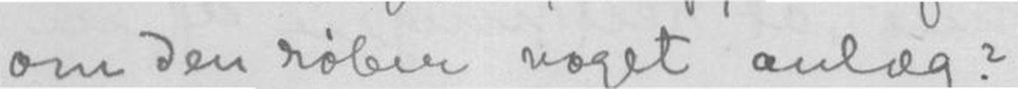om den røber noget anlæg?
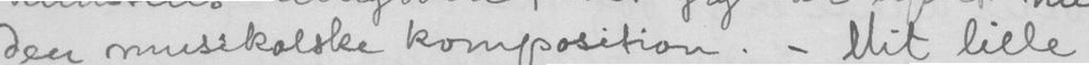den musikalske komposition. - Mit lille
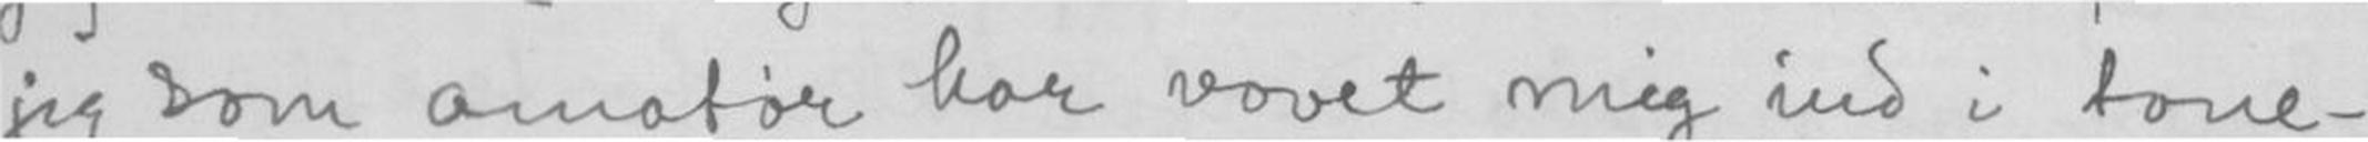jeg som amatør har vovet mig ind i tone-
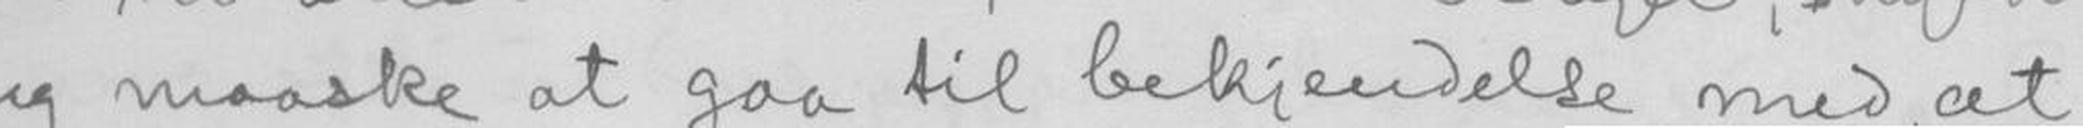jeg maaske at gaa til bekjendelse med, at
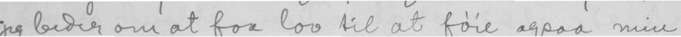jeg beder om at faa lov til at føie ogsaa min
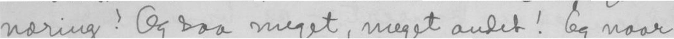næring! Og saa meget, meget andet! Og naar
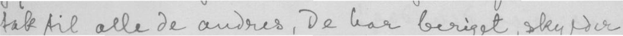tak til alle de andres, De har beriget, skylder
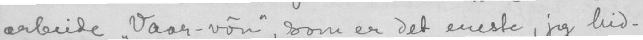arbeide Vaar-vón, som er det eneste, jeg hid-
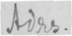Adrs.
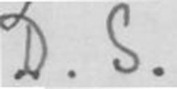D.S.
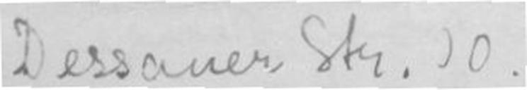Dessaner Str. 10.
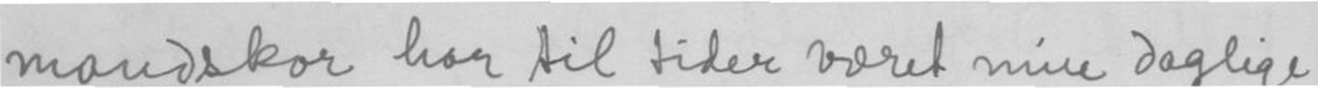mandskor har til tider været min daglige
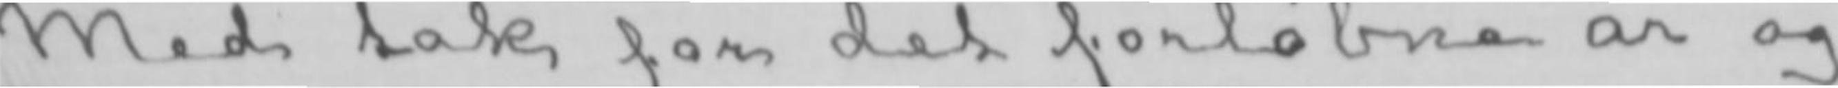Med tak for det forløbne år og
